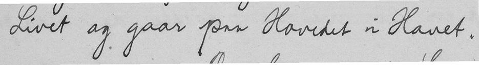Livet og gaar paa Hovedet i Havet.
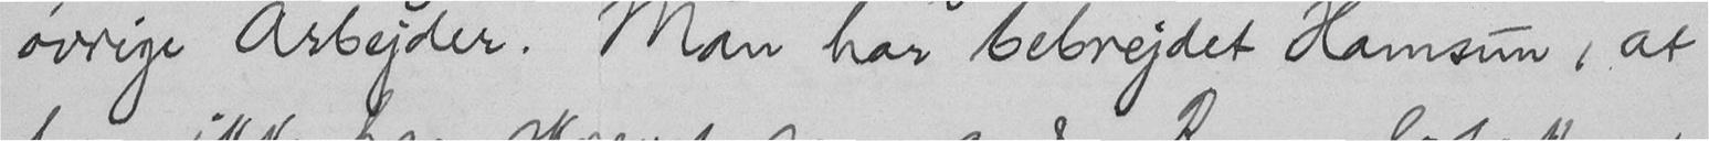øvrige Arbejder. Man har bebrejdet Hamsun, at
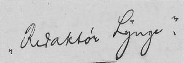"Redaktør Lynge".
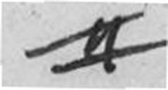#
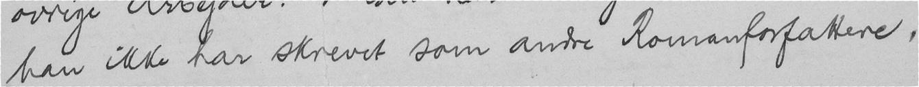han ikke har skrevet som andre Romanforfattere,
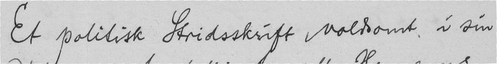Et politisk Stridsskrift voldsomt i sin
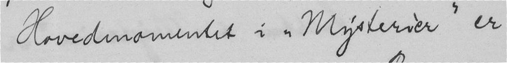Hovedmomentet i "Mysterier" er
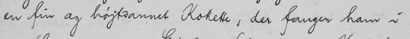en fin og højtdannet Kokette, der fanger ham i
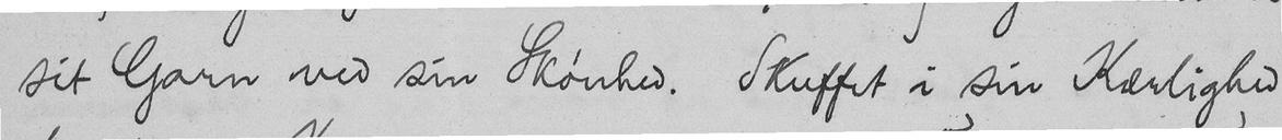sit Garn ved sin Skønhed. Skuffet i sin Kærlighed
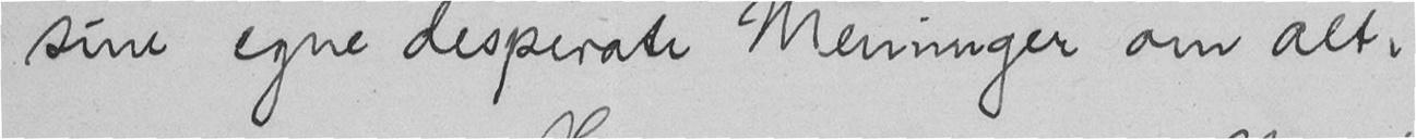sine egne desperate Meninger om alt.
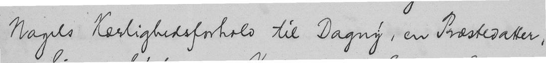Nagels Kærlighedsforhold til Dagny, en Præstedatter,
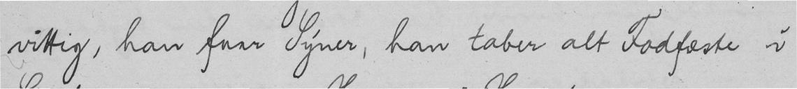vittig, han faar Syner, han taber alt Fodfæste i
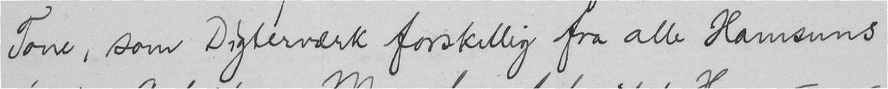Tone, som Digterværk forskellig fra alle Hamsuns
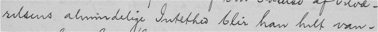relsens almindelige Intethed blir han helt van-
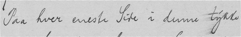Paa hver eneste Side i denne tykke
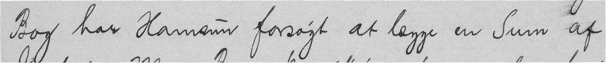Bog har Hamsun forsøgt at lægge en Sum af
